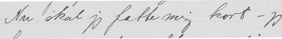Nu skal jeg fatte mig kort – jeg
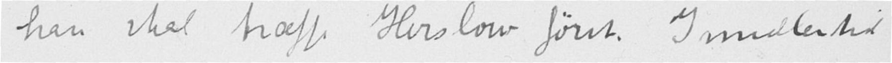han skal træffe Herslow først. Imidlertid
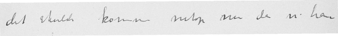det skulde komme netop nu da vi har
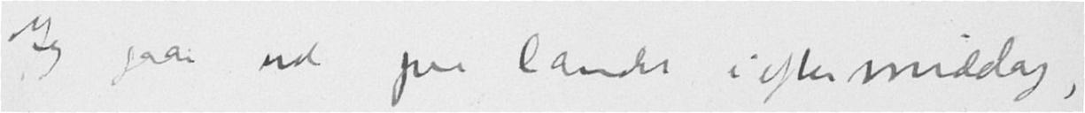Jeg gaar ud paa landet i eftermiddag,
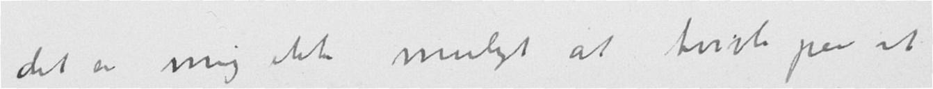det er mig ikke muligt at tviste paa at
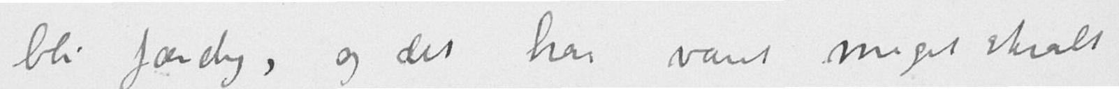bli færdig, og det har været meget skralt
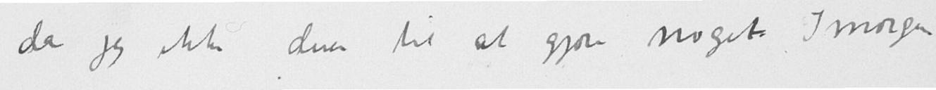da jeg ikke duer til at gjøre noget. I morgen
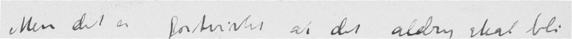Men det er fortvistet at det aldrig skal bli
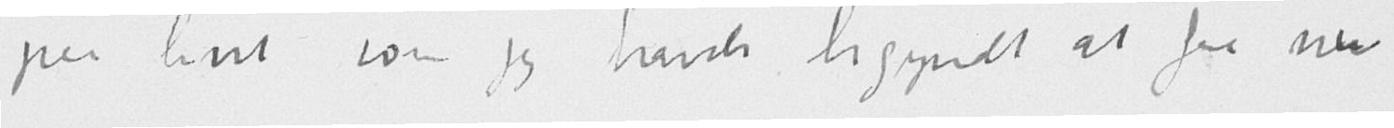paa livet som jeg havde begyndt at faa nu
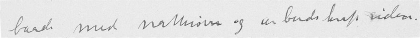baade med nattesøvn og arbeidskraft siden.
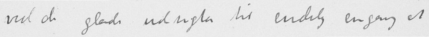ved de glade udsigter til endelig engang at
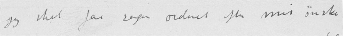jeg skal faa sagen ordnet efter mit ønske.
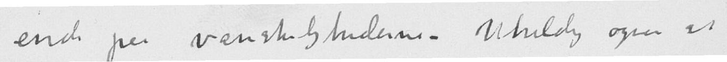ende paa vanskelighederne. Uheldig ogsaa at
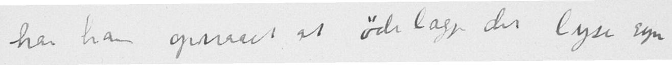har han opnaaet at ødelægge det lyse syn
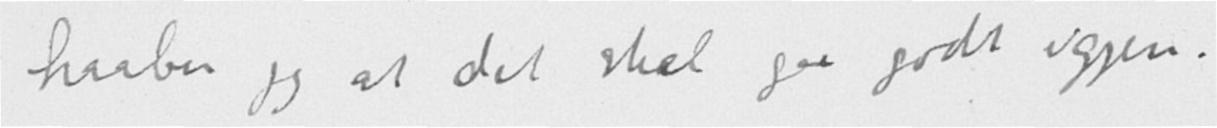haaber jeg at det skal gaa godt igjen.
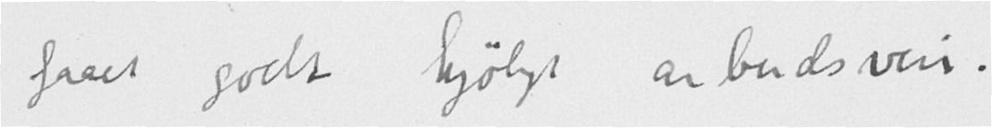faaet godt kjøligt arbeidsveir.
